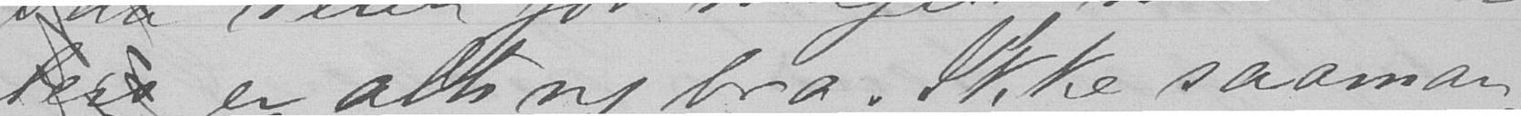lers er alting bra. Ikke saaman-
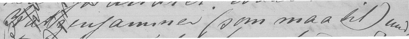Katzenjammer (som maa til) und-
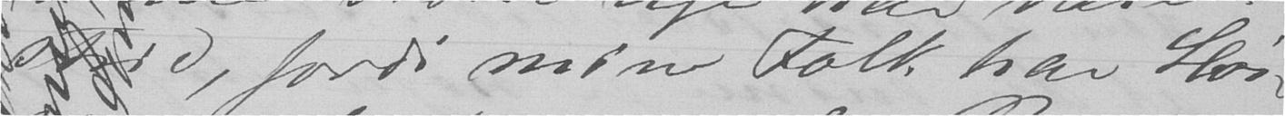strid, fordi mine Folk har Høi-
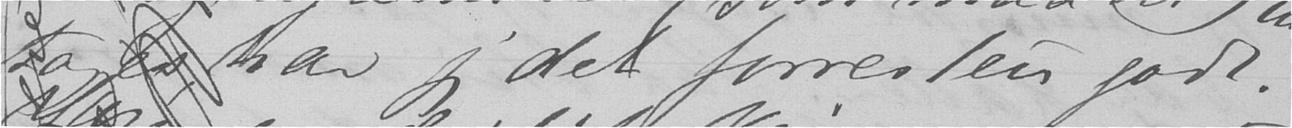tages, har jeg det forresten godt.
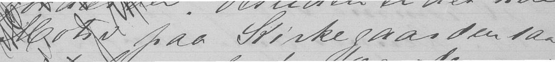Motiv paa Kirkegaarden saa
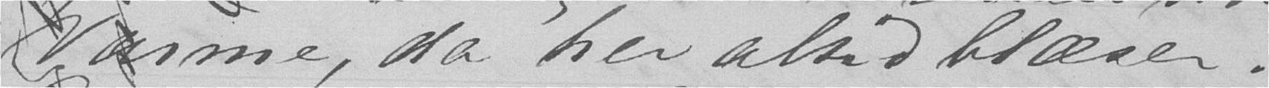Varme, da her altid blæser.
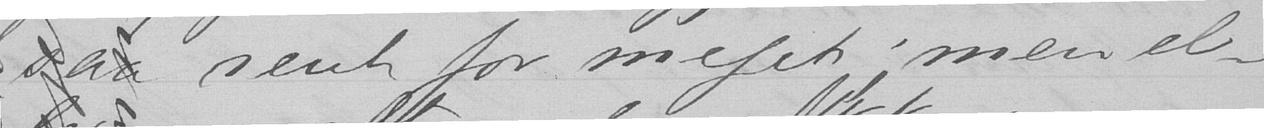saa rent for meget; men el-
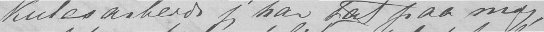kulesarbeide jeg har tat paa mig,
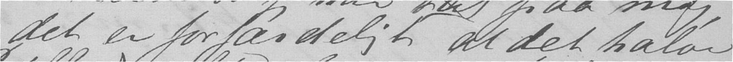det er forfærdeligt at det halve
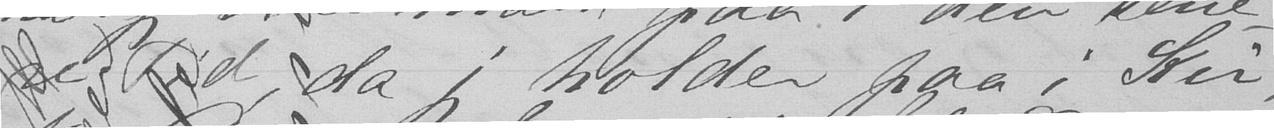re Tid, da jeg holder paa i Kir-
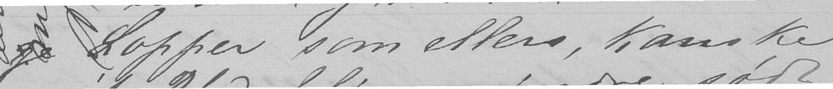ge Lopper som ellers, kanske
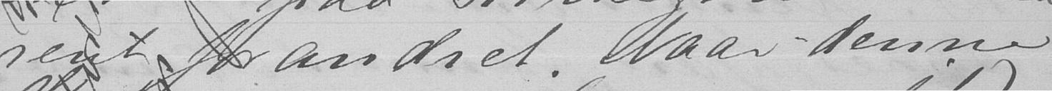rent forandret. Naar denne
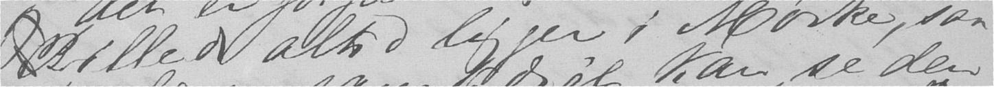Billede altid ligger i Mørke, saa
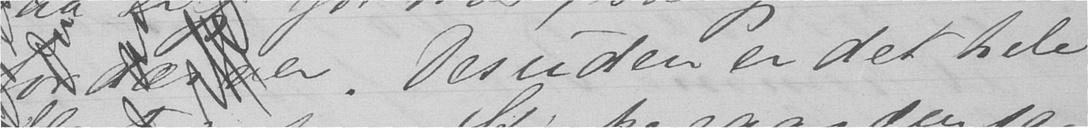fordærver. Desuden er det hele
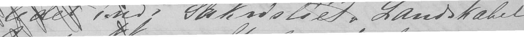ledet ind i Sakristiet. Landskabet
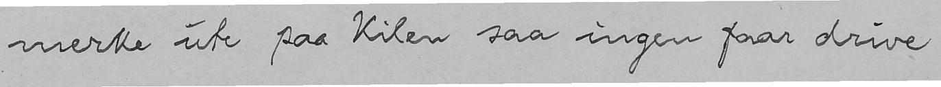merke ute paa Kilen saa ingen faar drive
